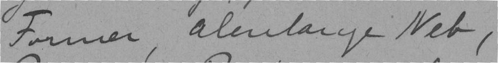Former, alenlange Neb,
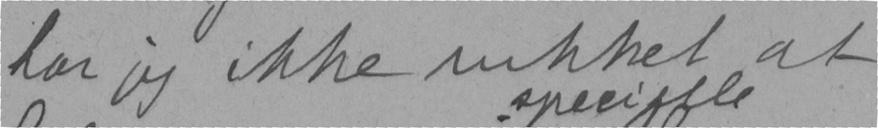har jeg ikke rukket at
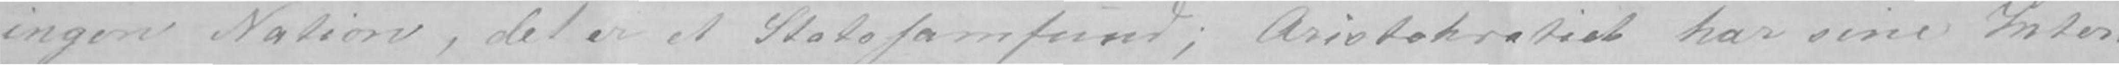ingen Nation, det er et Statssamfund; Aristokratiet har sine Inter-
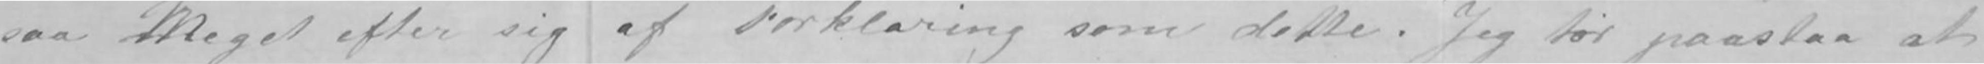saa Megetmeget efter sig af Forklaring som dette. Jeg tør paastaa at
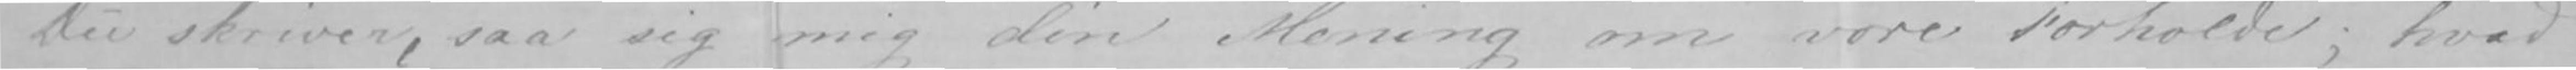Du skriver, saa sig mig din Mening om vore Forholde; hvad
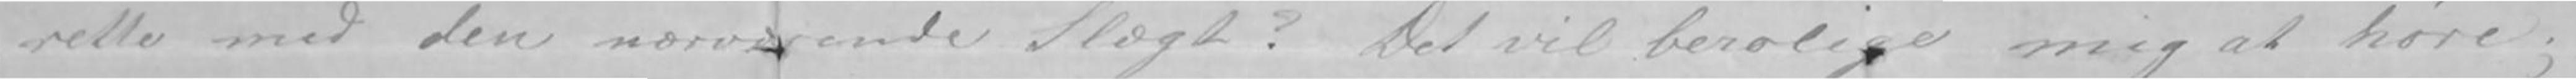rette med den nærværende Slægt? Det vil berolige mig at høre;
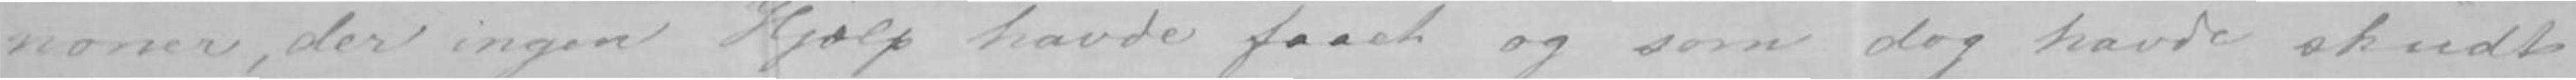noner, der ingen Hjælp havde faaet og som dog havde skudt
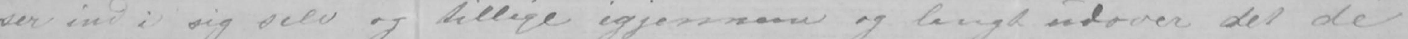ser ind i sig selv og tillige igjennem og langt udover det de
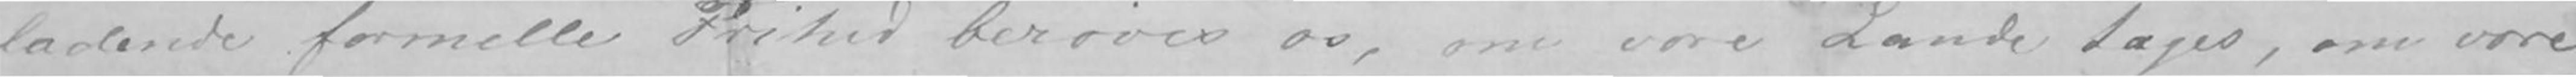ladende formelle Frihed berøves os, om vore Lande tages, om vore
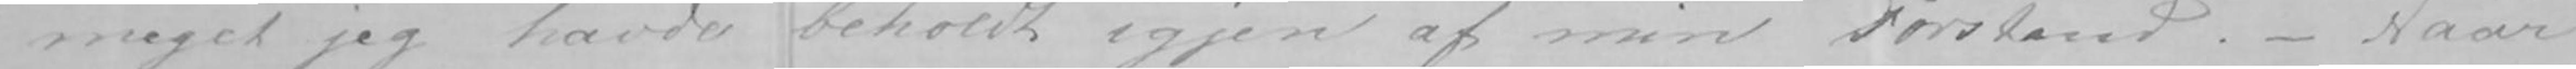meget jeg havde beholdt igjen af min Forstand.- Naar
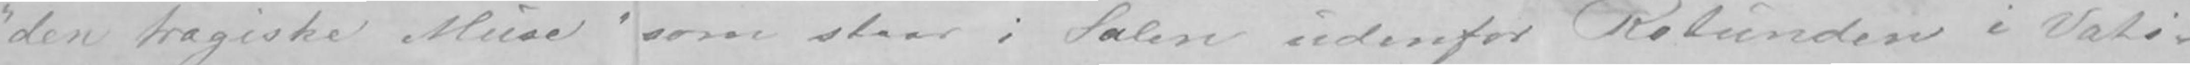«den tragiske Muse» som staar i Salen udenfor Rotunden i Vati-
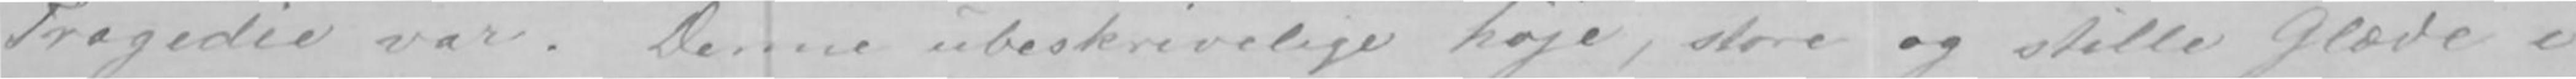Tragedie var. Denne ubeskrivelige høje, store og stille Glæde i
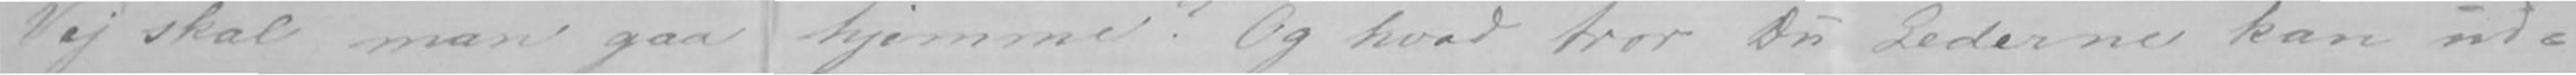Vej skal man gaa hjemme? Og hvad tror Du Lederne kan ud-
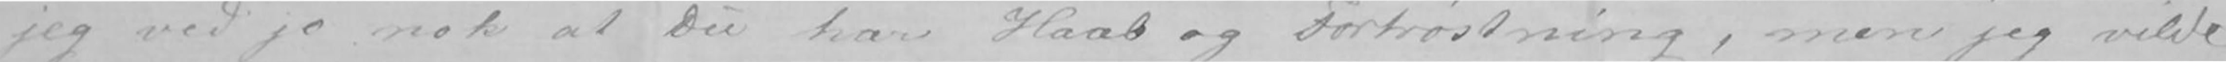jeg ved jo nok at Du har Haab og Fortrøstning, men jeg vilde
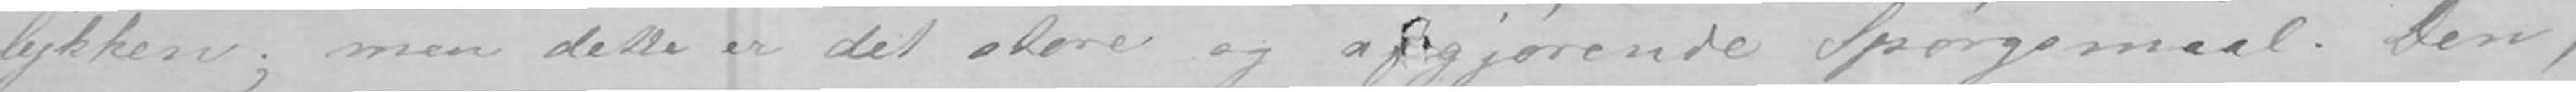lykken; men dette er det store og afgjørende Spørgsmaal. Den,
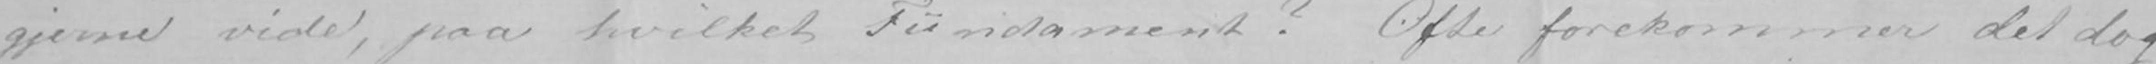gjerne vide, paa hvilket Fundament? Ofte forekommer det dog
